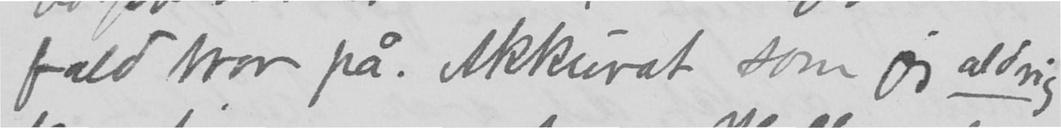fald tror fra. Akkurat, som jeg aldrig
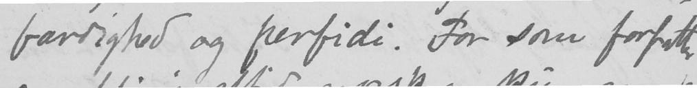færdighed og perfidi. For som forfatter
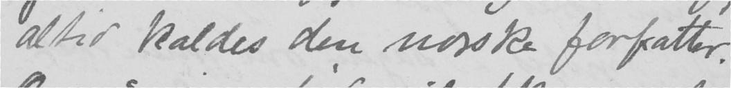altid kaldes den norske forfatter.
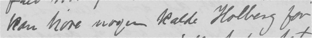kan høre nogen kalde Holberg for
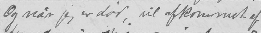Og når jeg er død vil afkommet af
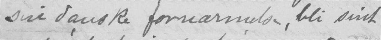sin danske fornærmelse, bli sint
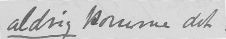aldrig komme det
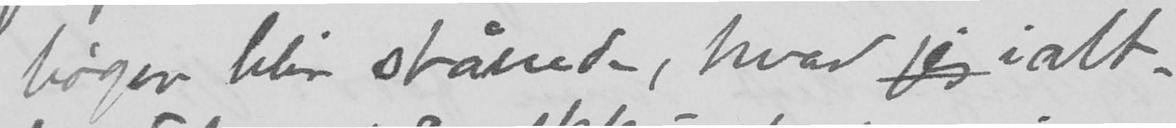bøger blir stående, hvad jeg ialt-
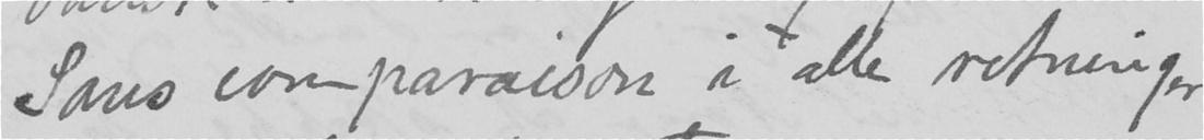Sans comparaison i alle retninger,
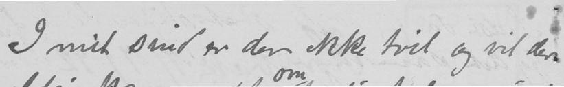I mit sind er der ikke tvil og vel der
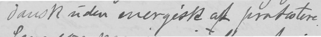dansk uden energisk at protestere.
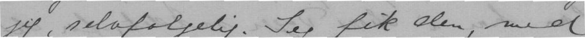jeg, selvfølgelig. Jeg fik den, med
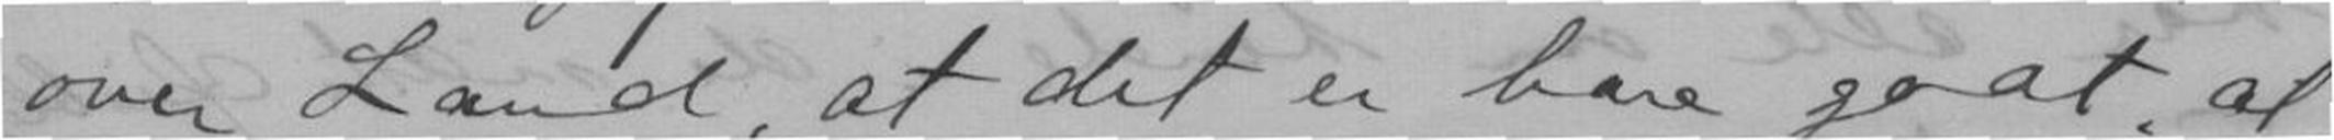over Land, at det er bare godt at
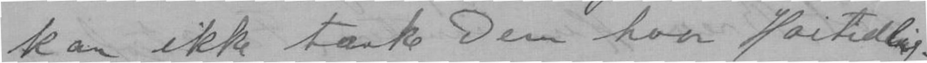kan ikke tænke Dem hvor Høitidlig-
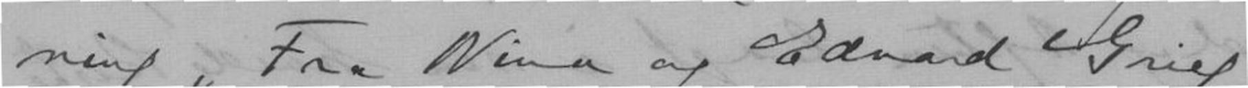ning "Fra Nina og Edvard Grieg"
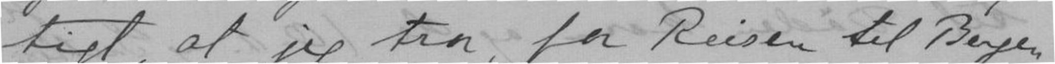tigt, at jeg tror, for Reisen til Bergen
